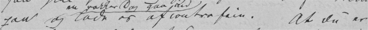paa og og lade os afcontrafeie. At Du er
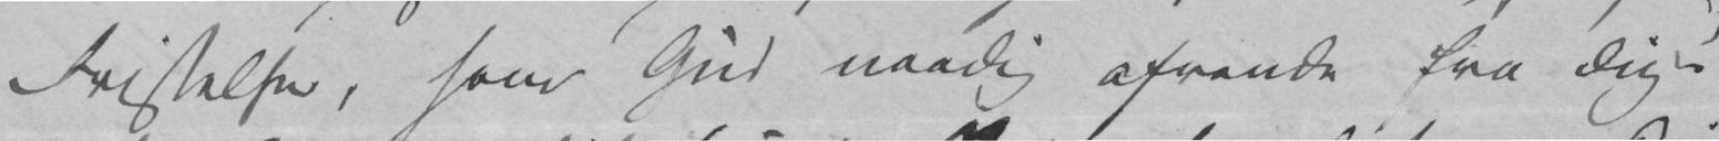Fristelser, som Gud naadig afvende fra Dig!
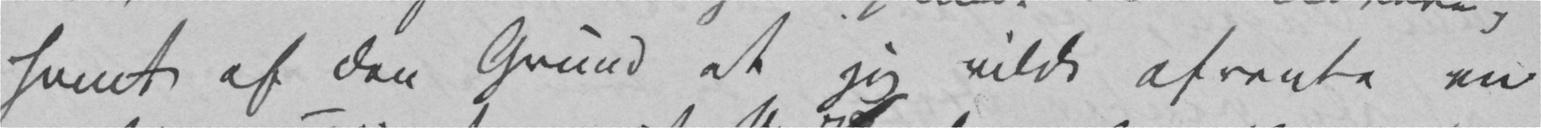samt af den Grund at jeg vilde afvente en
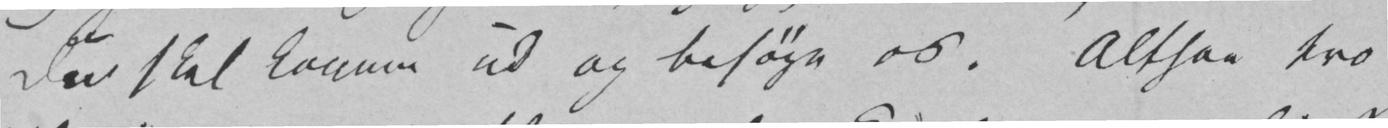Du skal komme ud og besøge os. Altsaa tro
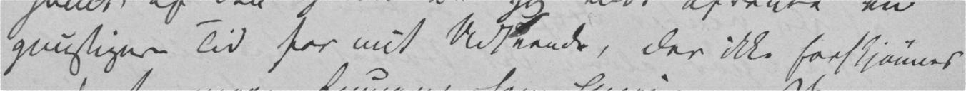gunstigere Tid for mit Udseende, der ikke forskjønnes
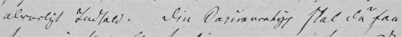alvorligt Indhold. Din Daguerrotyp skal Du faa
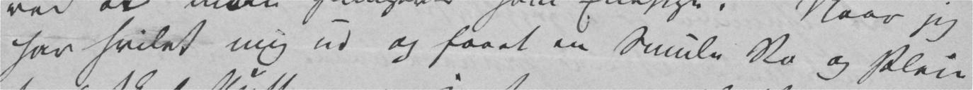har hvilet mig ud og faaet en Smule Ro og Pleie
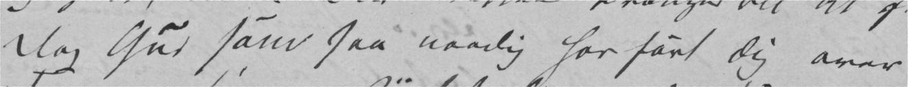Dog Gud som saa naadig har ført Dig over
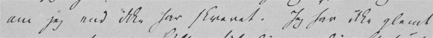om jeg end ikke har skrevet. Jeg har ikke glemt
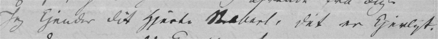Jeg kjender Dit Hjerte Robert, det er kjerligt.
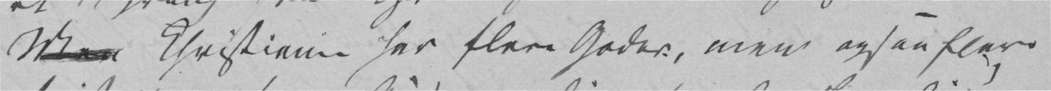Men Christiania har flere Goder, men ogsaa flere
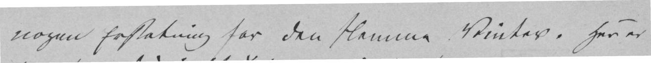nogen Erstatning for den slemme Vinter. Her er
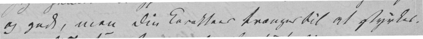og godt, men Din Carakteer trænger til at styrkes.
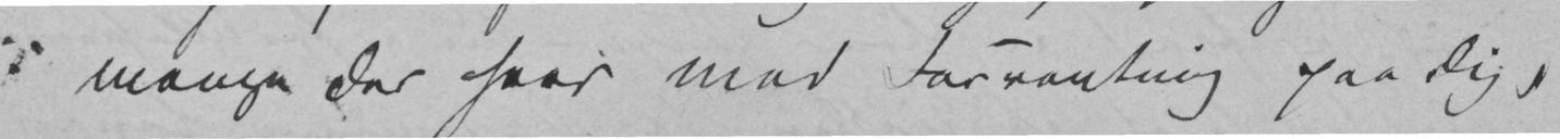mange der seer med Forventning paa Dig,
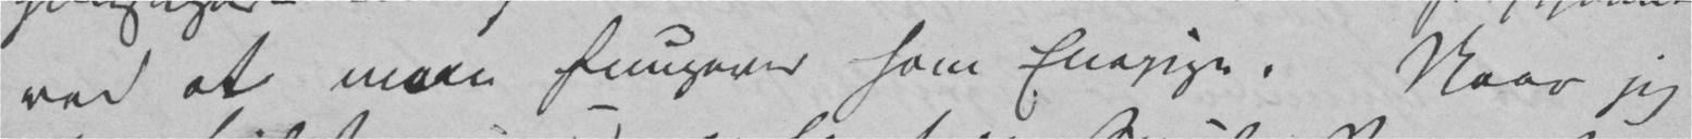ved at man fungerer som Enepige. Naar jeg
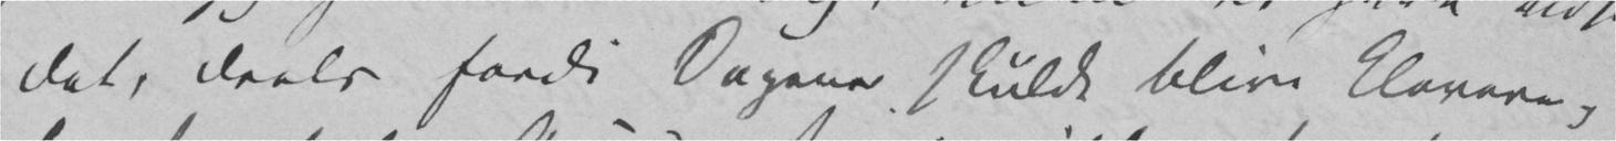det, deels fordi Dagene skulde blive klarere,
